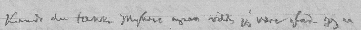Kunde du takke Myhre ogsaa vilde jeg være glad. Jeg er
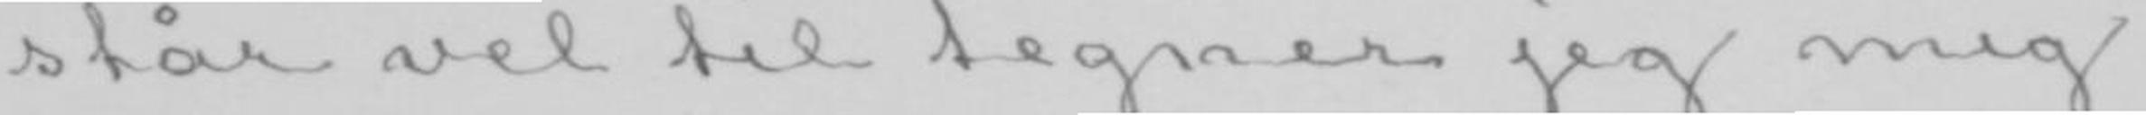står vel til tegner jeg mig
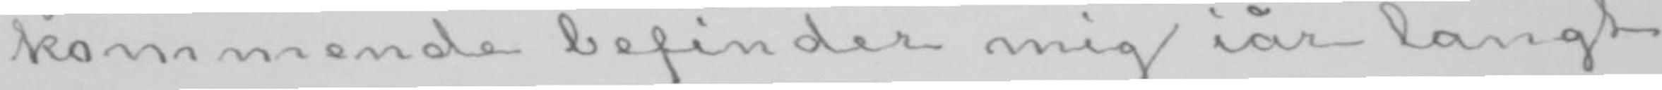kommende befinder mig iår langt
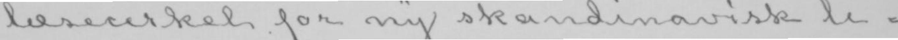læsecirkel for ny skandinavisk li-
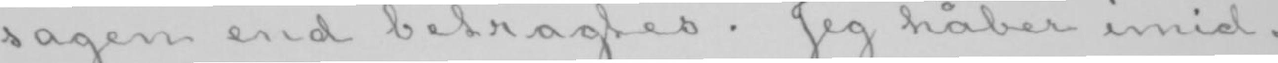sagen end betragtes. Jeg håber imid-
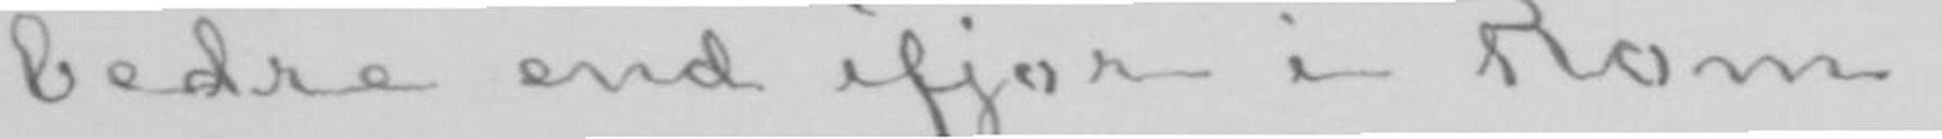bedre end ifjor i Rom.
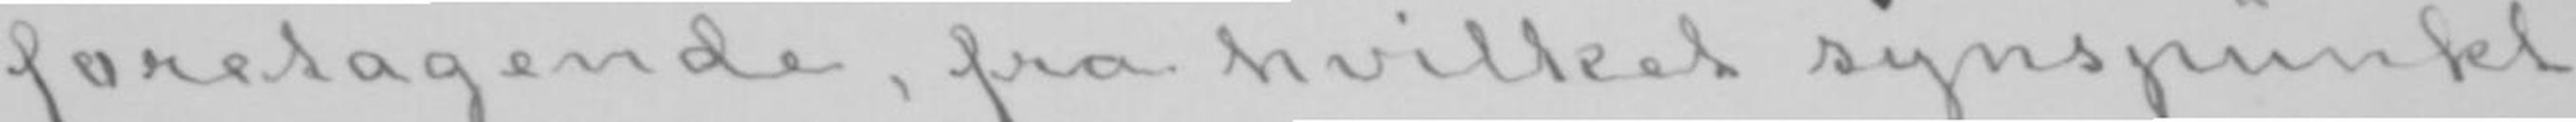foretagende, fra hvilket synspunkt
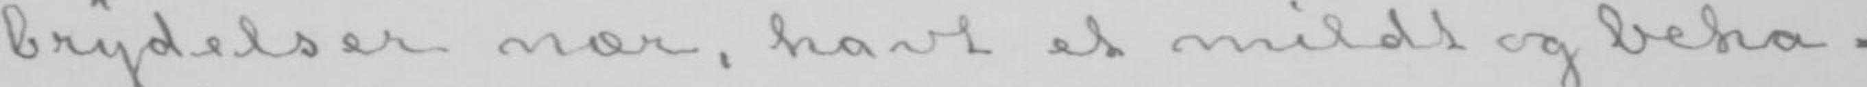brydelser nær, havt et mildt og beha-
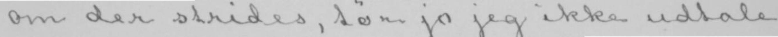om der strides, tør jo jeg ikke udtale
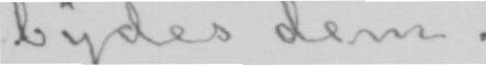bydes dem.
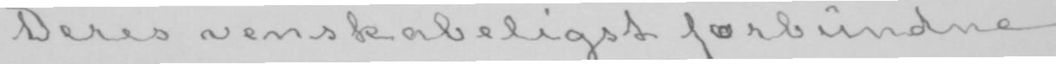Deres venskabeligst forbundne
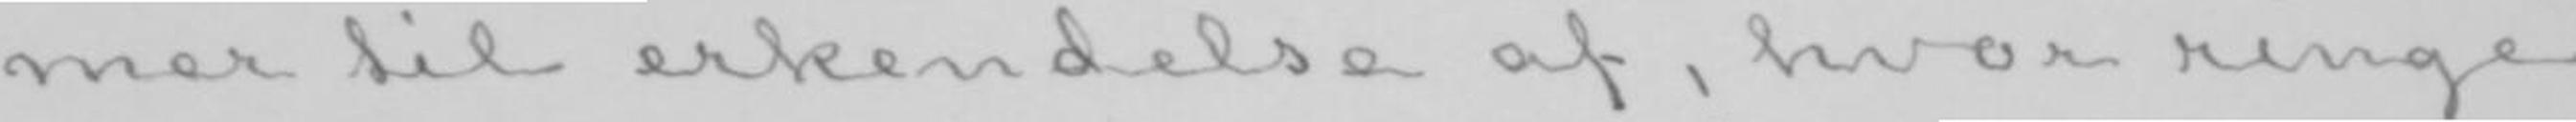mer til erkendelse af, hvor ringe
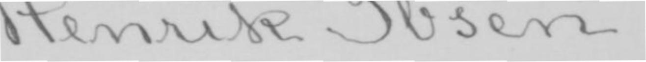Henrik Ibsen.
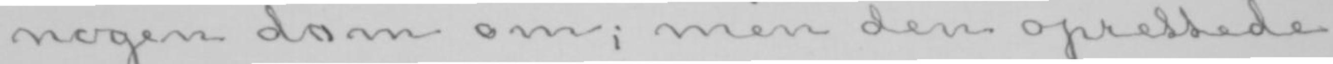nogen dom om; men den oprettede
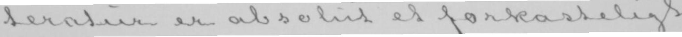teratur er absolut et forkasteligt
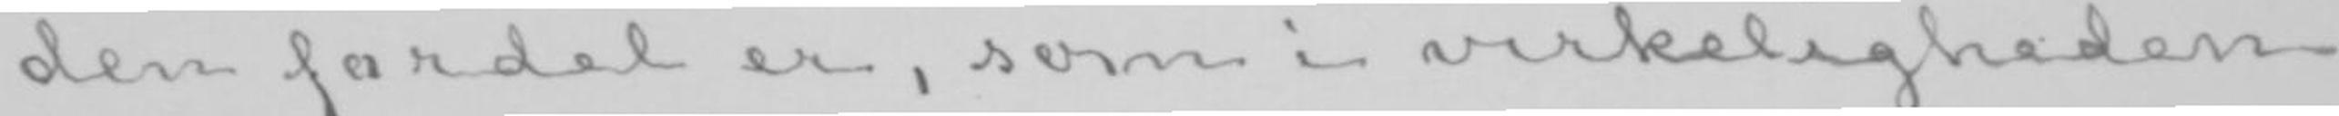den fordel er, som i virkeligheden
